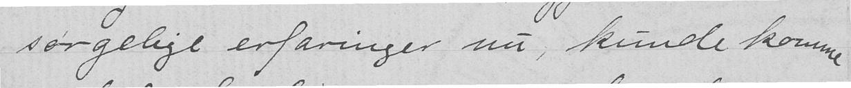sørgelige erfaringer nu, kunde komme
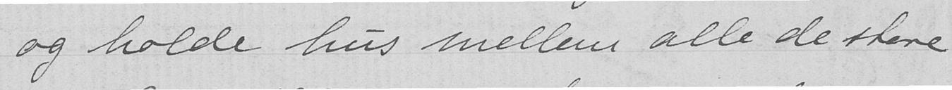og holde hus mellem alle de store
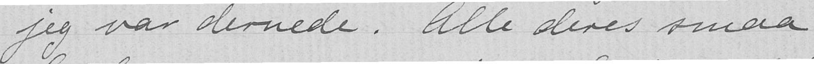jeg var dernede. Alle deres smaa
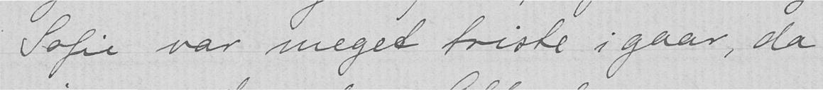Sofie var meget triste i gaar, da
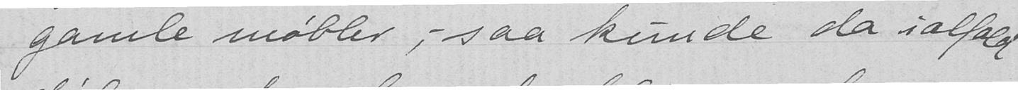gamle møbler, - saa kunde da ialfald
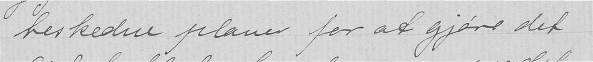beskedne planer for at gjøre det
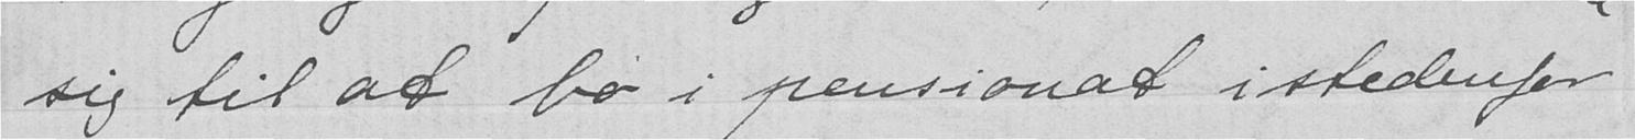sig til at bo i pensionat istedenfor
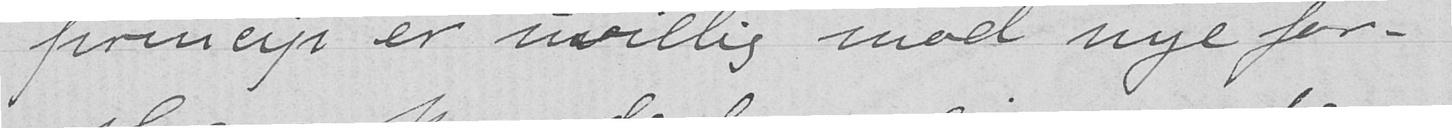princip er uvillig mod nye for-
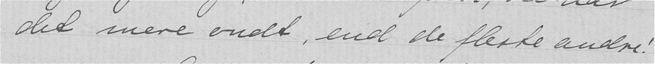det mere ondt, end de fleste andre!
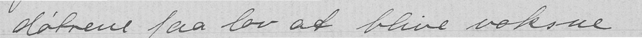døtrene faa lov at blive voksne
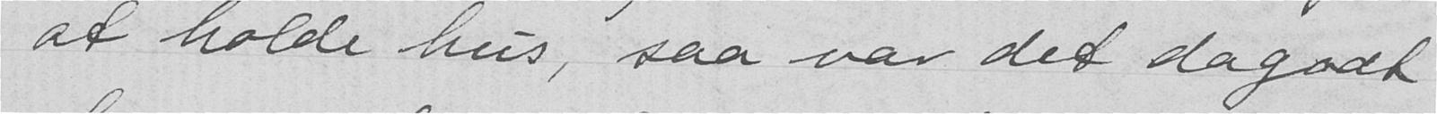at holde hus, saa var det da godt
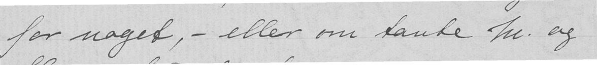for noget, - eller om tante M. og
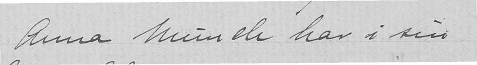Anna Munch har i sin
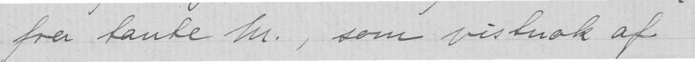fra tante M., som vistnok af
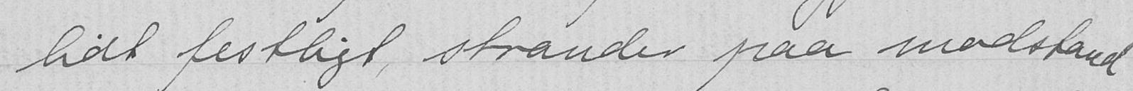lidt festligt, strander paa modstand
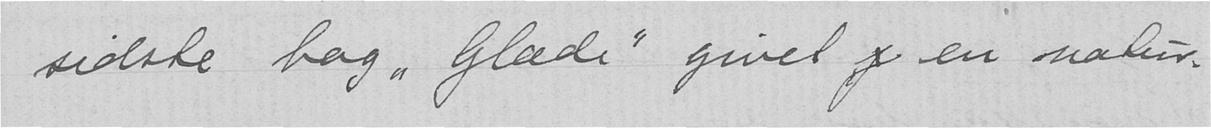sidste bog "Glæde" givet en natur-
